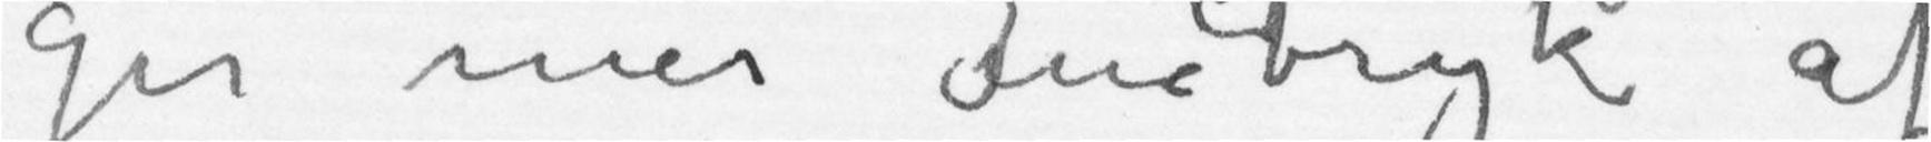gir mer iIndtryk af
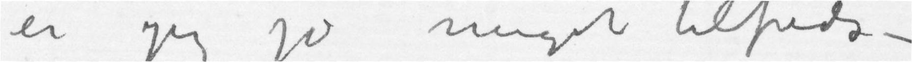er jeg jo meget tilfreds –
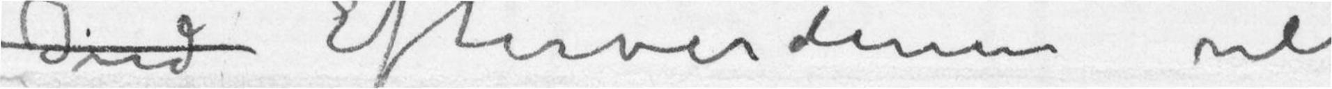Ind Efterverdenen vil
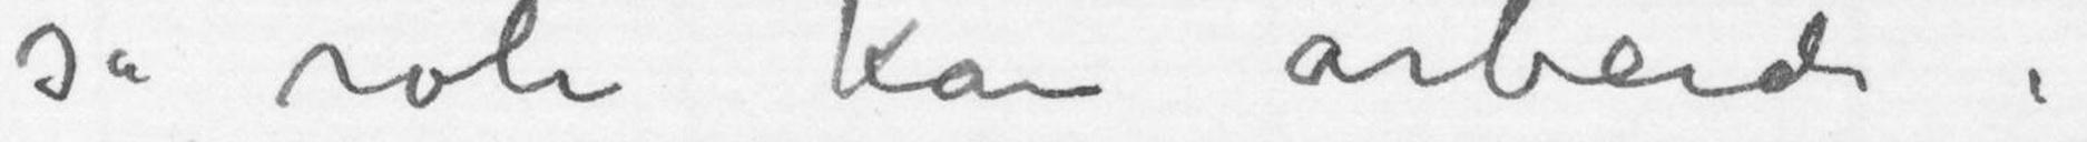så roli kan arbeide i
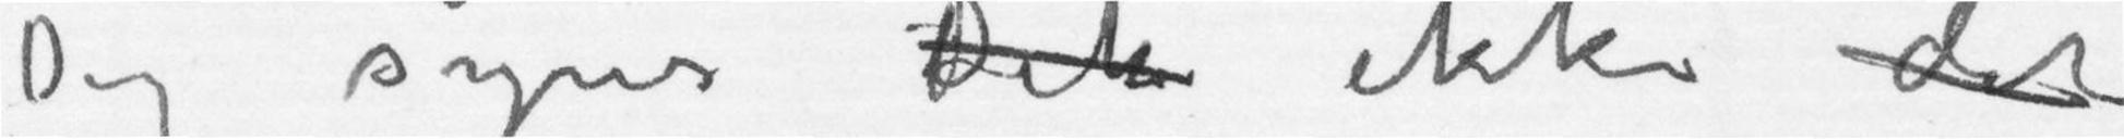Dog synes D ikke det
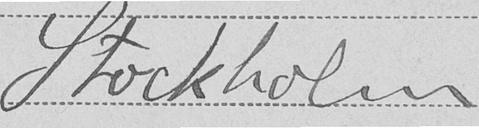Stockholm
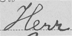Herr
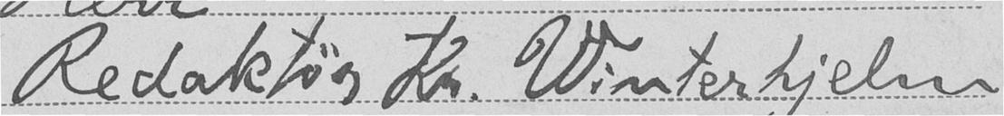Redaktør Kr. Winterhjelm
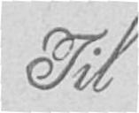Til
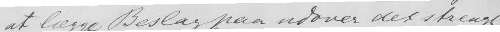at lægge Beslag paa udover det strengt
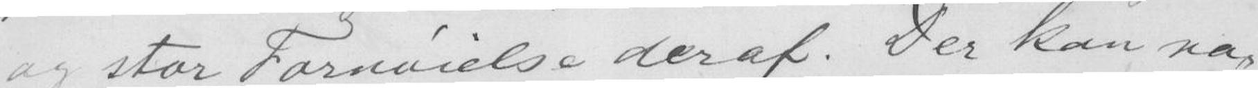og stor Fornøielse deraf. Der kan na
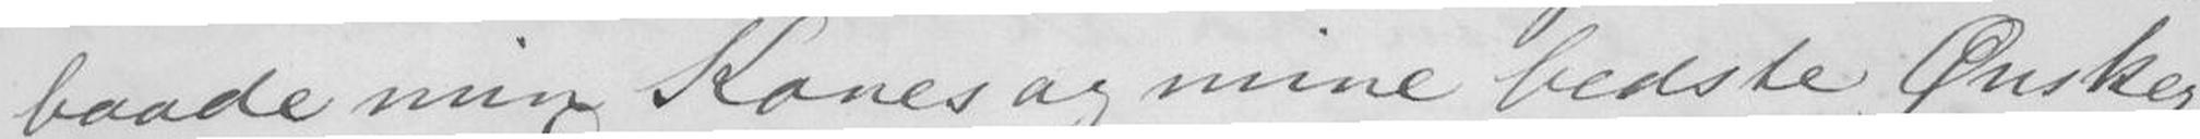baade min Kones og mine bedste Ønsker
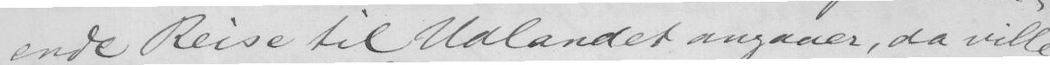ende Reise til Udlandet Angaar, da ville
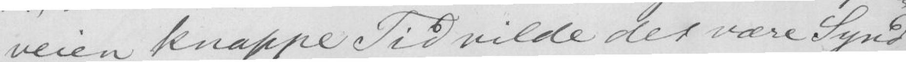veien knappe Tid vilde det være Synd
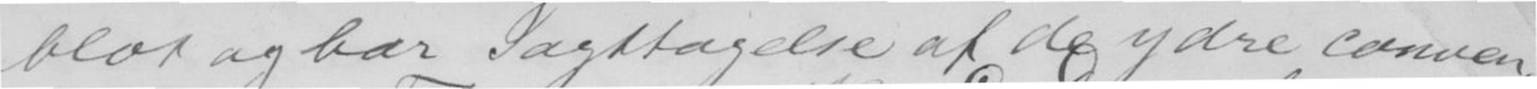blot og bar Iagtagelse af de ydre conven
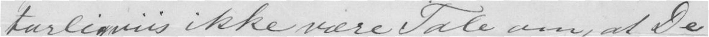turligviis ikke være Tale om, at De
